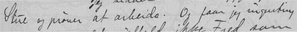Stue og prøver at arbeide. Og faar jeg ingenting
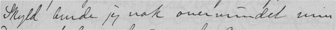Skyld burde jeg nok overvundet min
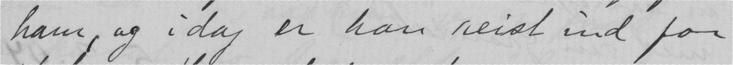ham, og i dag er han reist ind for
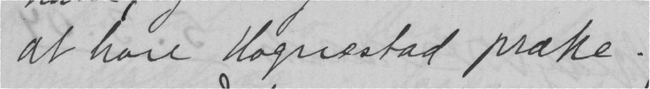at høre Hognestad præke.
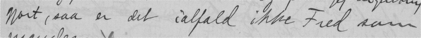gjort, saa er det ialfald ikke Fred som
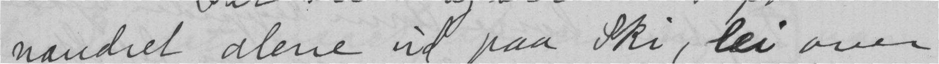vandret alene ud paa Ski, lei over
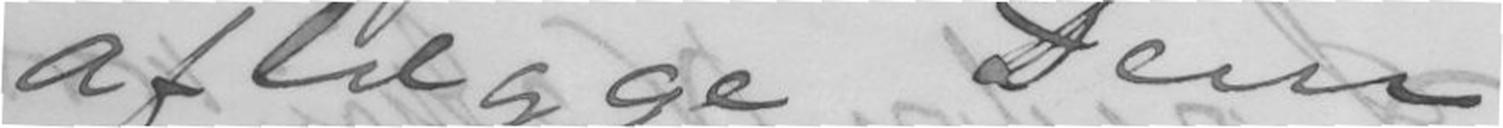aflægge Dem
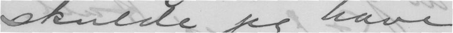skulde jeg have
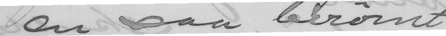en saa berømt
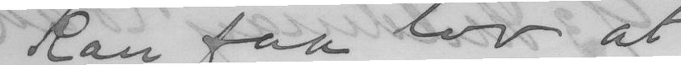kan faa lov at
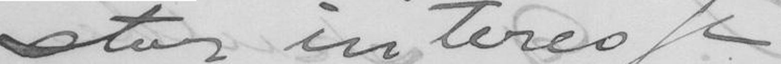stor interesse
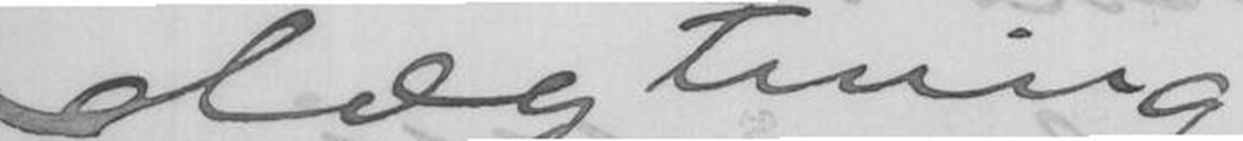slægtning.
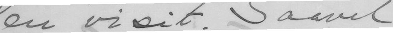en visit. Saavel
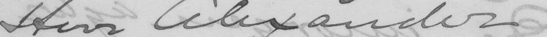Herr Alexander
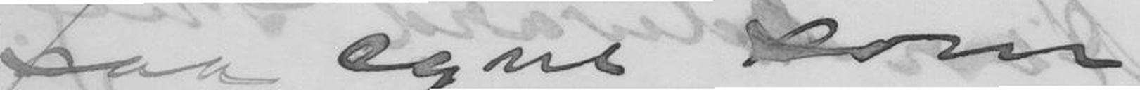paa egne som
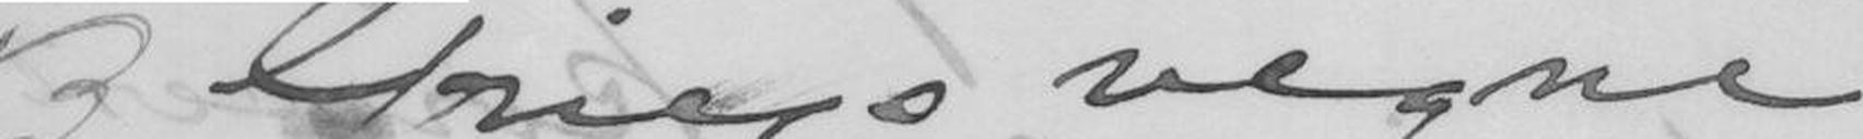B Griegs vegne
